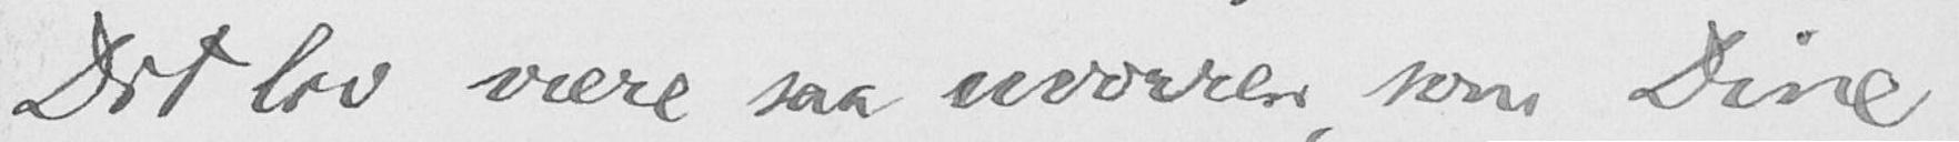Dit liv være saa uvorren som Dine
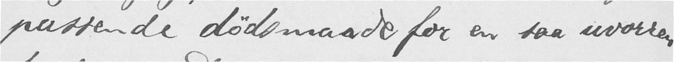passende dødsmaade for en saa uvorren
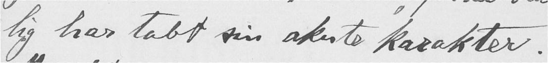lig har tabt sin akute karakter.
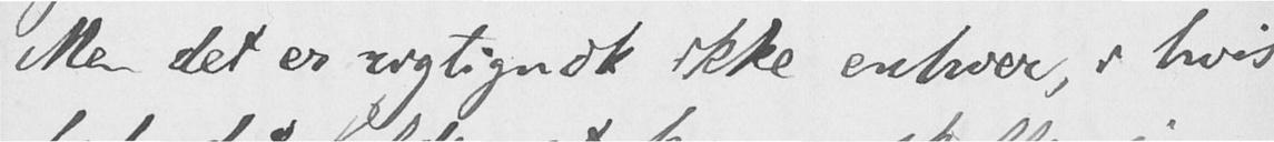Men det er rigtignok ikke enhver, i hvis
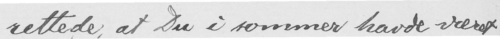rettede, at Du i sommer havde været
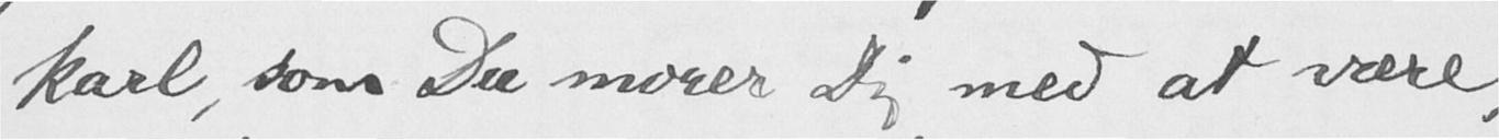karl, som Du morer Dig med at være,
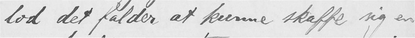lod det falder, at kunne skaffe sig en
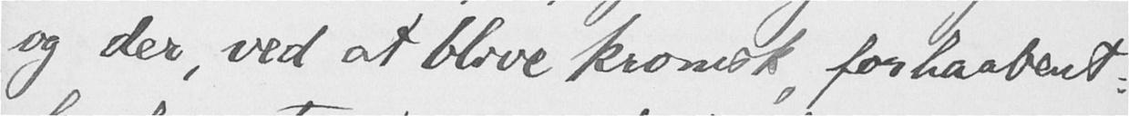og der, ved at blive kronisk, forhaabent-
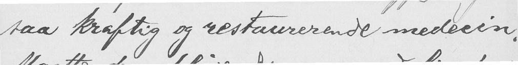saa kraftig og restaurerende medecin.
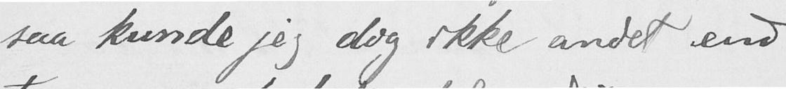saa kunde jeg dog ikke andet end
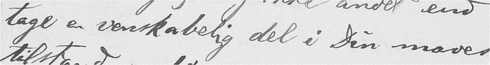tage en venskabelig del i Din maves
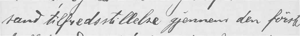sand tilfredsstillelse gjennem den første
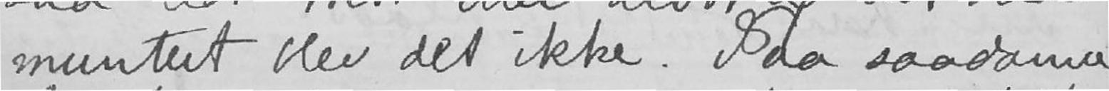muntert blev det ikke. Paa saadanne
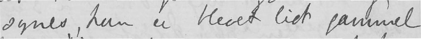synes, hun er blevet lidt gammel
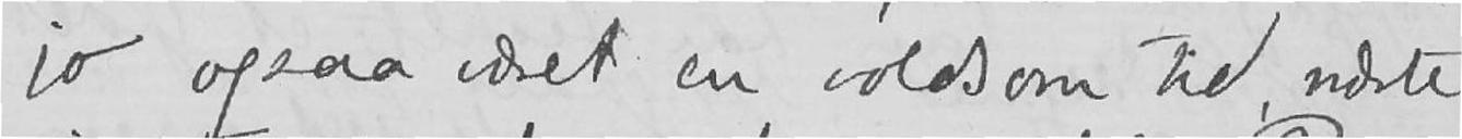jo ogsaa været en voldsom tid, næste
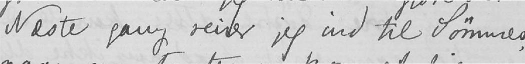Næste gang reiser jeg ind til Sømmes,
